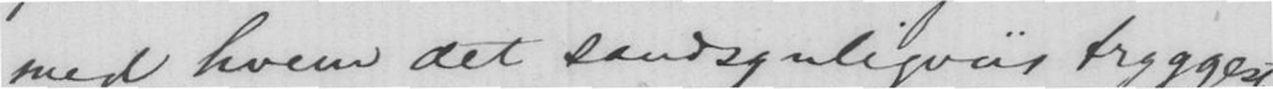med hvem det sandsynligvis tryggest
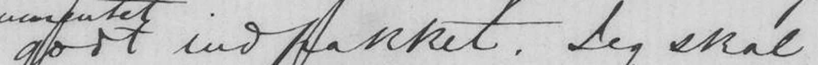godt indpakket. Jeg skal
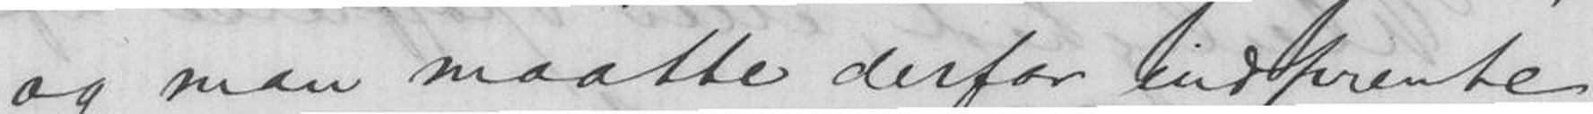og man maatte derfor indprente
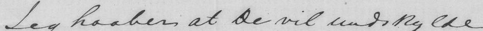Jeg haaber at De vil undskylde
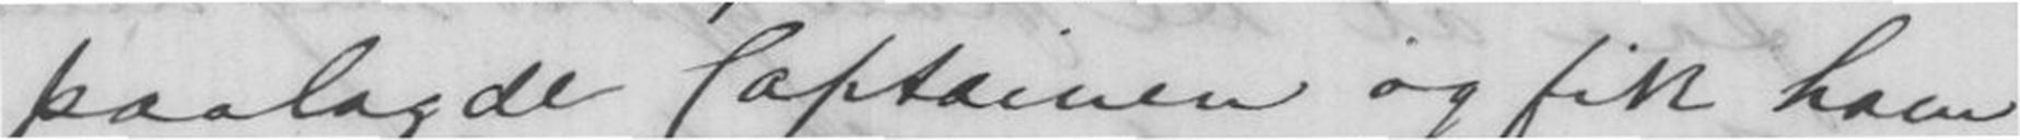paalagde Captainen og fik ham
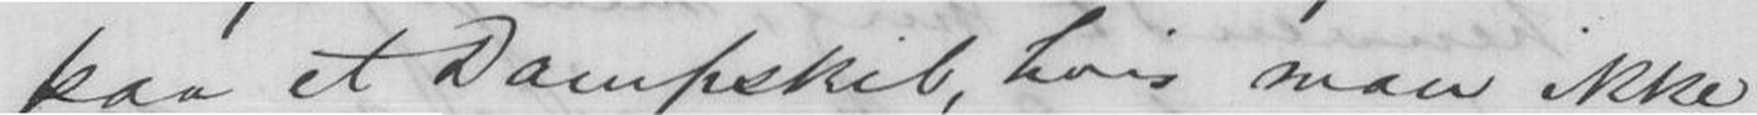paa et Dampskib, hvis man ikke
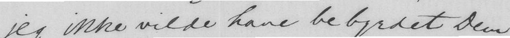jeg ikke vildee have bebyrdet Dem
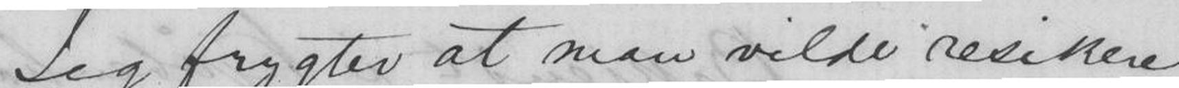Jeg frygter at man vilde resikere
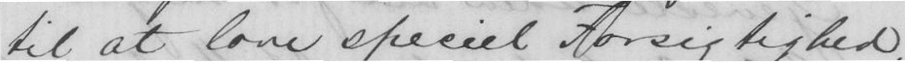til at love speciel Forsigtighed,
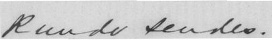kunde sendes.
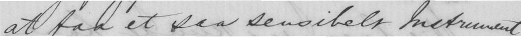at faa et saa sensibelt Instrument
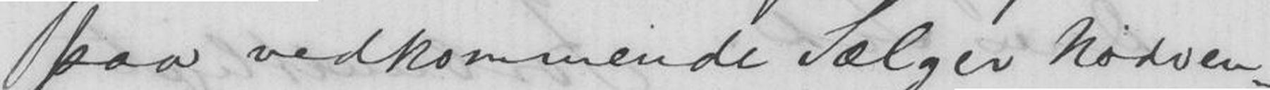paa vedkommende Sælger Nødven-
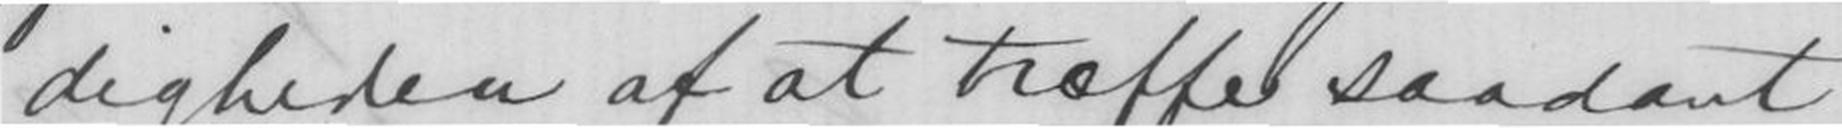digheden af at træffe saadant
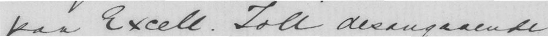paa Exell. Toll desangaaende,
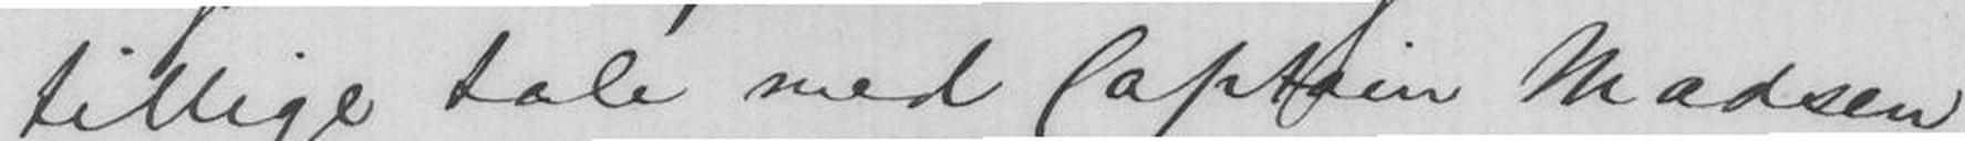tillige tale med Captain Madsen
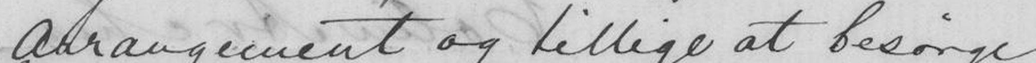Arrangement og tillige at besørge
